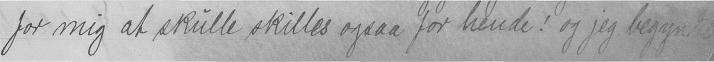for mig at skulle skilles ogsaa for hende! og jeg begyndte
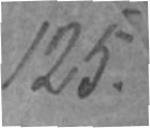125.
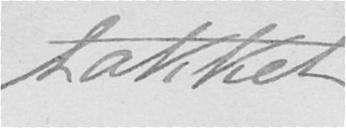takket
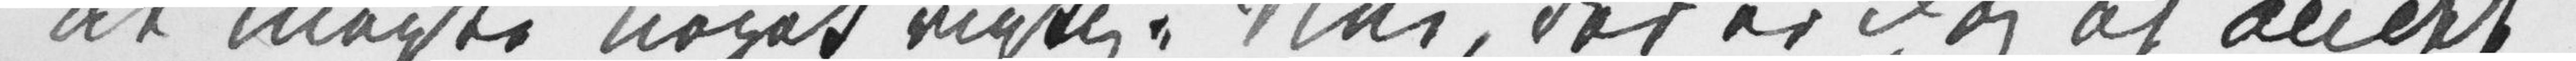at magte noget rigtig. Nei, der er dog af andet
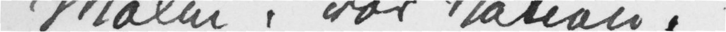Malm i vor Nation.
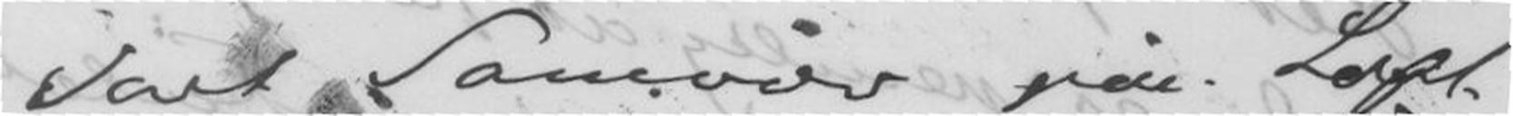Vårt Samvær påe Loft-
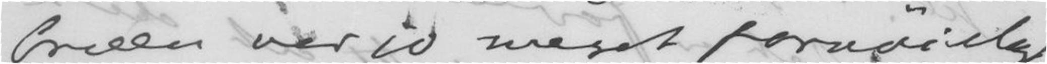bræen var jo meget fornøielig
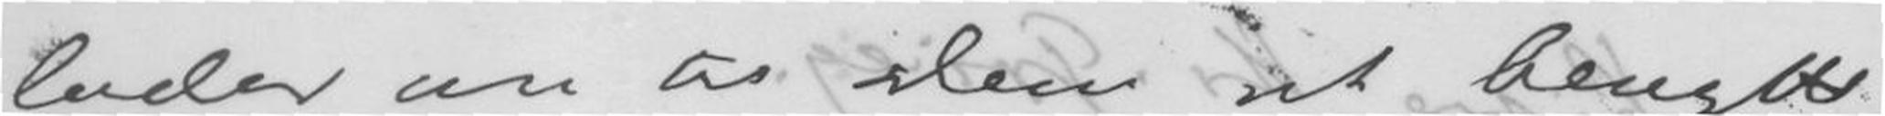lader nu til dem at benytte
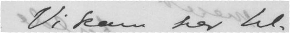Vi kom her til-
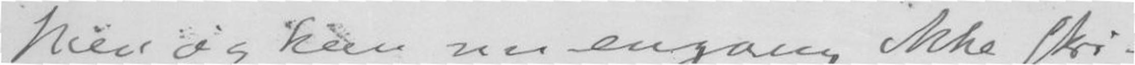Men os kan nu engang ikke skri-
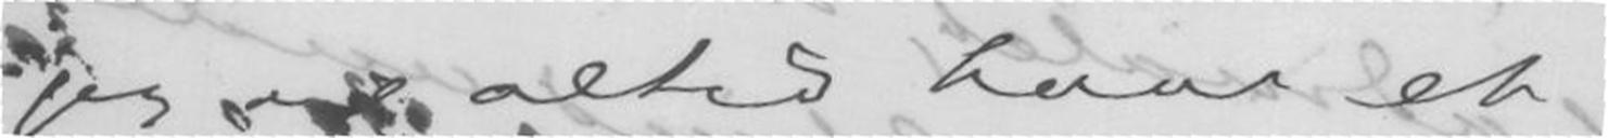jeg vil altid have et
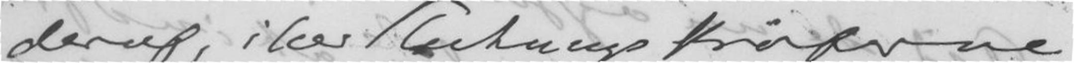deraf, især Slutnings stroferne.
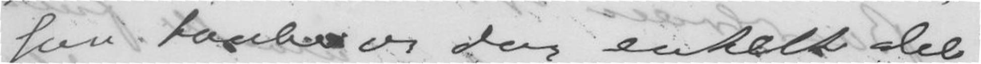saa tænker vi der enkelt del
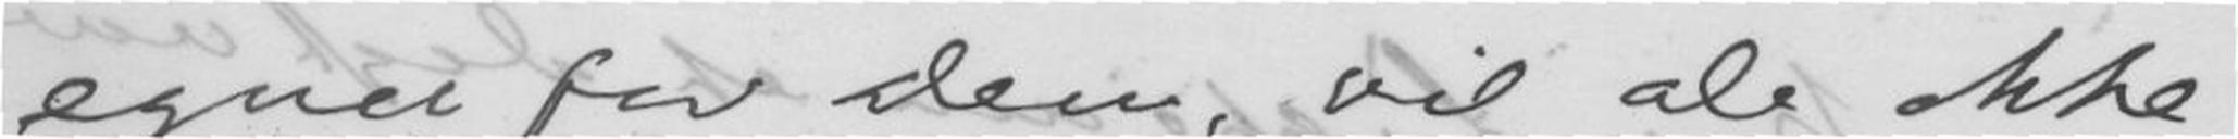egnet for Dem, vil De ikke
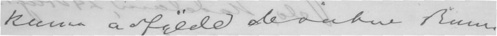kunne adfylde deaabne Rum.
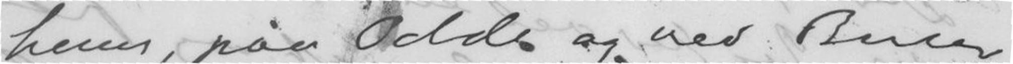hus, påe Odde og ved Buar
